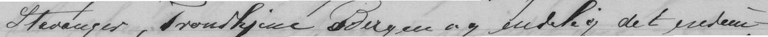Stavanger, Trondhjem, Bergen og endelig det endnu
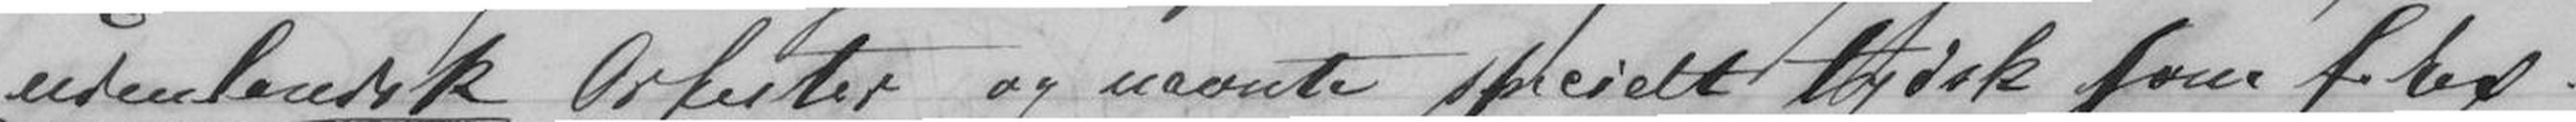udenlandsk Orkester og nævnte specielt tydsk som f Ex.
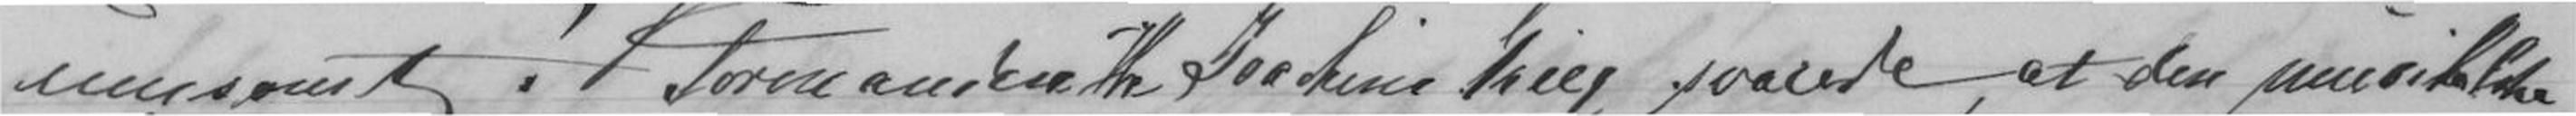uensomt! Formand Hr Joackim Grieg svarede, at den musikalske
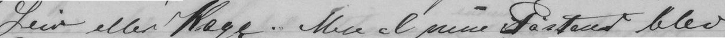Leiv eller Kage. Men al min Påstand blev
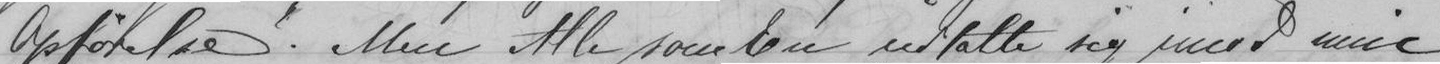Opførelse". Men Alle som En udtalte sig imod min
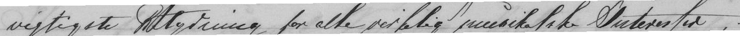vigtigste Betydning for alle virkelig musikalske Interessed, -
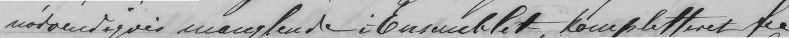nødvendigvis manglende i Ensemblet, kompletteret fra
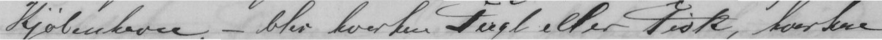Kjøbenhavn, - blir hverken Fugl eller Fisk, hverken
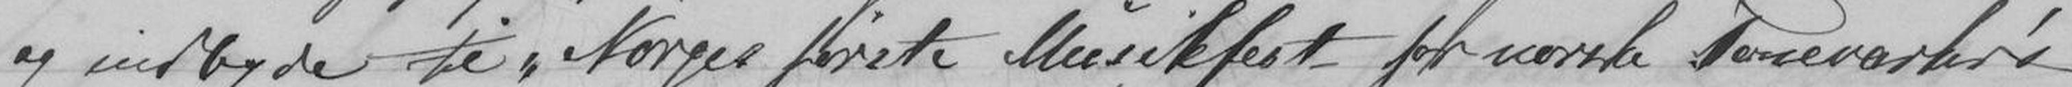og indbyde til "Norges første Musikfest for norske Toneværkers
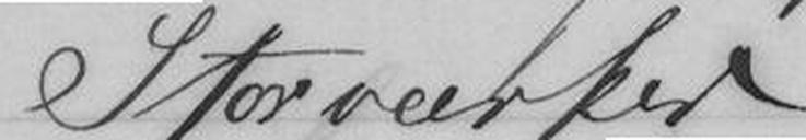Storværker.
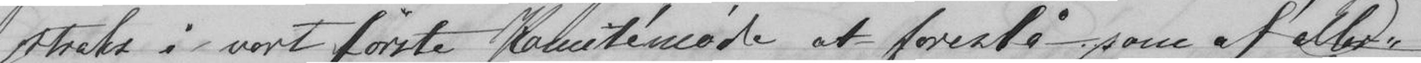straks i vort første Komitémøde at foreslå som af aller-
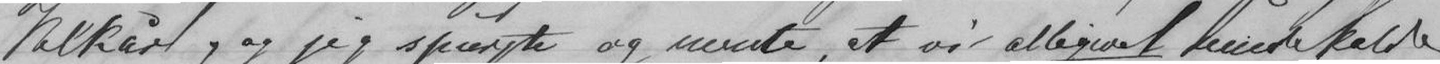Vilkår, og jeg spurgte og mente, at vi alligevel kunde kalde
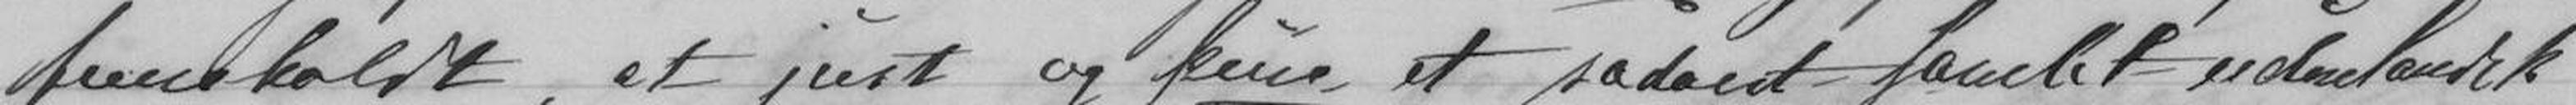fremholdt, at just og kun et sådant samlet udenlandsk
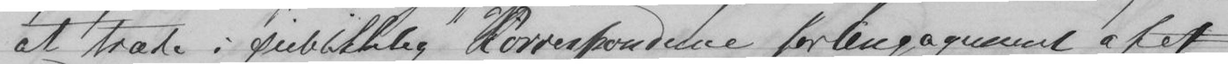at træde i Øieblikkeleg Korrespondance for Engagement af et
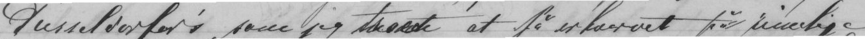Düsseldorfer's, som jeg troede at få erhvervet på rimelige
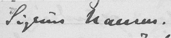Sigurd Hansen.
